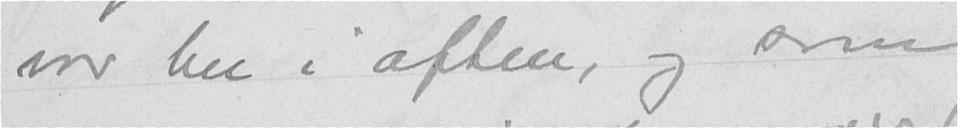var her i aften, og som
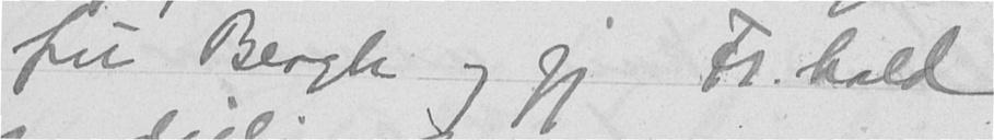fru Bergh og jeg Fr. hald
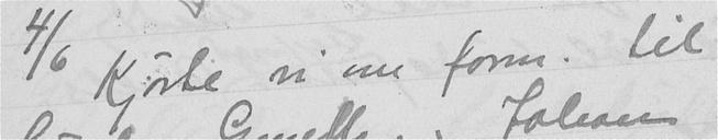4/6 Kjørte vi om form. til
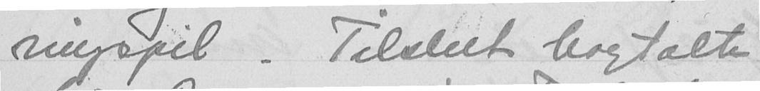ringspil. Tilslut bagtalte
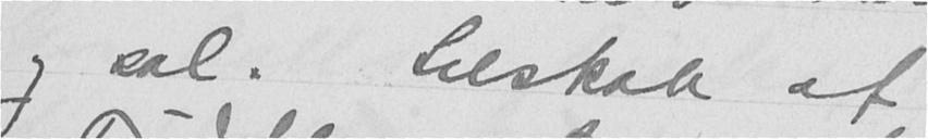og sol. Selskab af
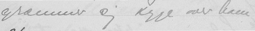græmmer sig syge over ham.
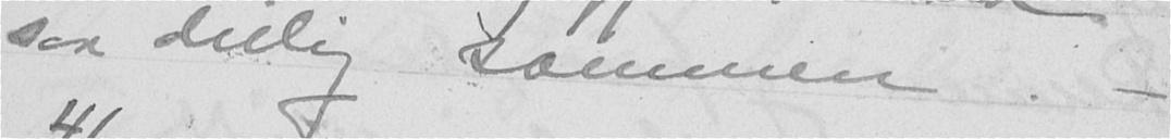saa deilig sammen. -
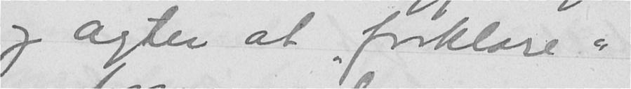og agter at "forklare"
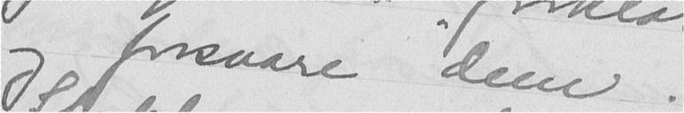og forsvare dem.
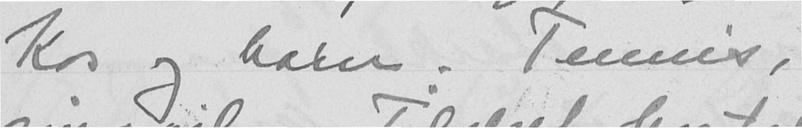Kos og barn. Tennis,
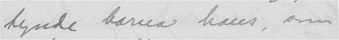tynde barna hans, som
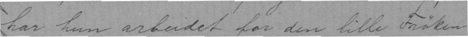har hun arbeidet for den lille Frøken
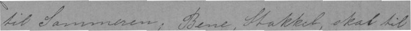til Sommeren; Bene, Stakket, skal til
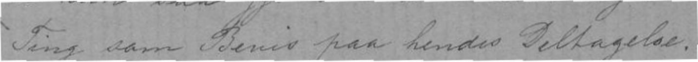Ting som Bevis paa hendes Deltagelse.
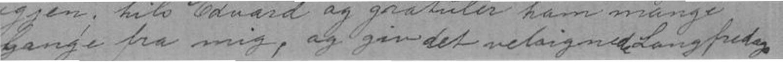Gange fra mig, og giv det velsignede Langfredags-
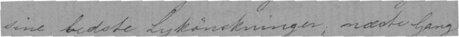sine bedste Lykønskninger; næste Gang
